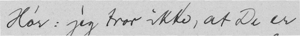Hør: jeg tror ikke, at De er
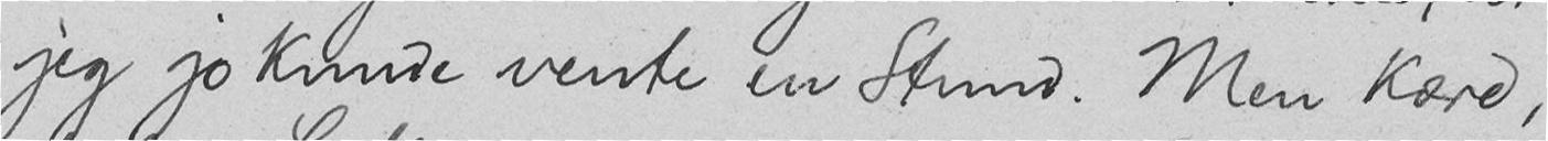jeg jo kunde vente en Stund. Men kære,
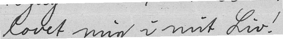lovet mig i mit Liv!
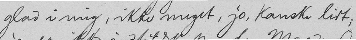glad i mig, ikke meget, jo, kanske lidt;
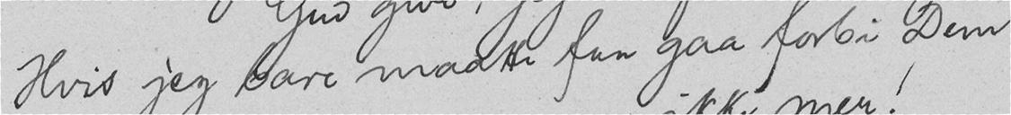Hvis jeg bare maatte faa gaa forbi Dem
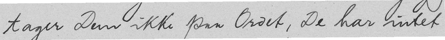tager Dem ikke paa Ordet, De har intet
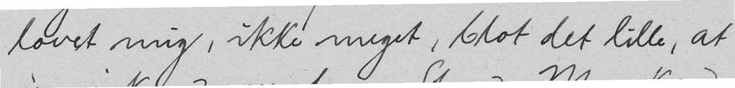lovet mig, ikke meget, blot det lille, at
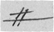#
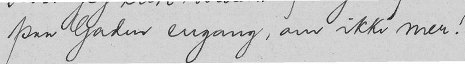paa Gaden engang, om ikke mer!
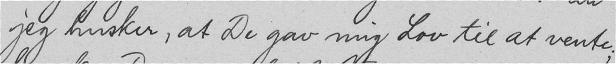jeg husker, at De gav mig Lov til at vente;
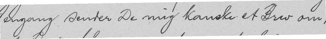engang sender De mig kanske et Brev om,
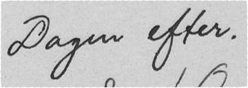Dagen efter.
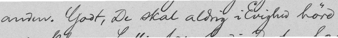anden. Godt, De skal aldrig i Evighed høre
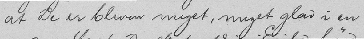at De er bleven meget, meget glad i en
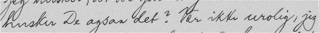husker De ogsaa det? Vær ikke urolig, jeg
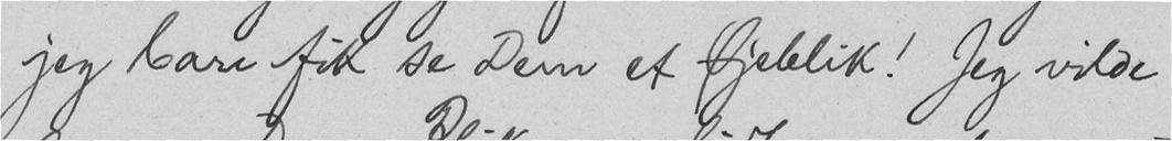jeg bare fik se Dem et Øjeblik! Jeg vilde
